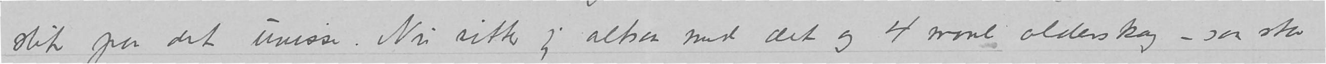slik paa det uvisse. Nu sitter jeg altsaa med det og 4 maal olderskog – saa stor
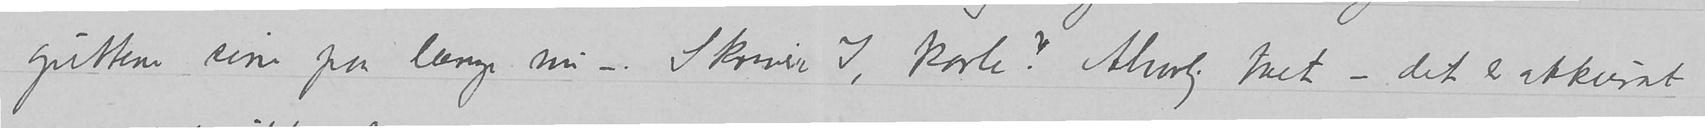guttene sine paa længe nu -. Skrivere 7, korte! Alvorlig taet – det er ikkurat
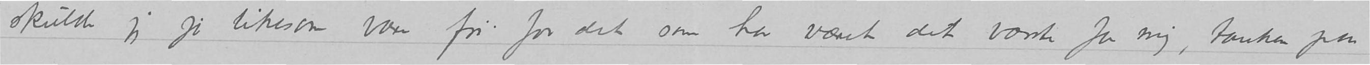skulde jeg jo likesom være fri for det som har været det vonde  for mig, tanken paa
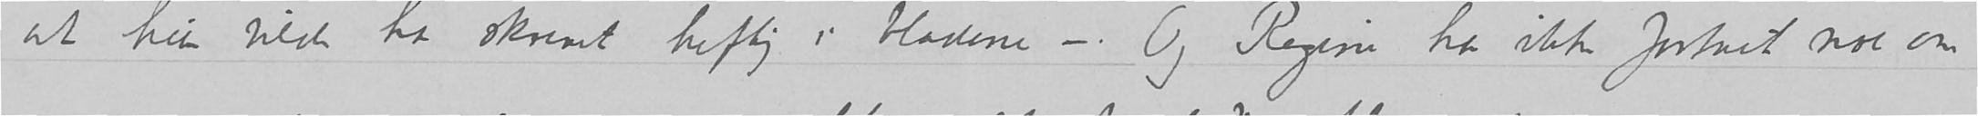at hun vilde ha skrevet heftig i bladene –. Og Regine har ikke fortalt noe om
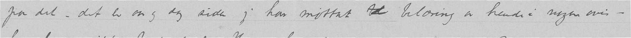paa det – det er aar og dag siden jeg har mottat te belæring av hende i nogen avis –
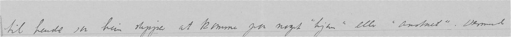til hende saa hun slipper at komme paa noget «hjem» eller «anstalt». Dermed
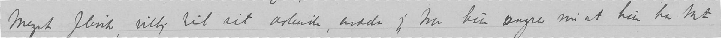Meget flink, villig til sit arbeide, endda jeg tror hun ængrer nu at hun har tat
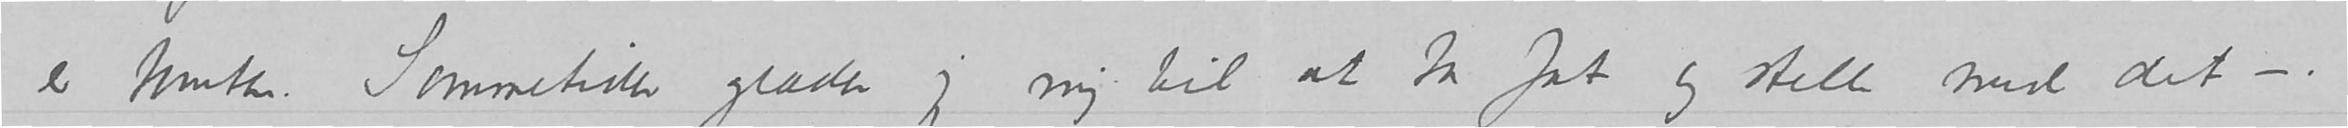er tomten. Sommetider glæder jeg mig til at ta fat og stelee med det –.
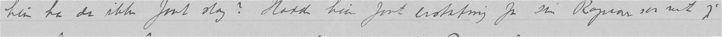hun har da ikke faat slag? Hadde hun faat erstatnig for sin Rapiov saa vet jeg
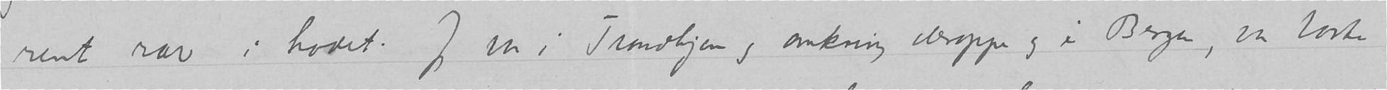rent rar i hodet. Jeg var i Trondhjem og omkring deroppe og i Bergen, var borte
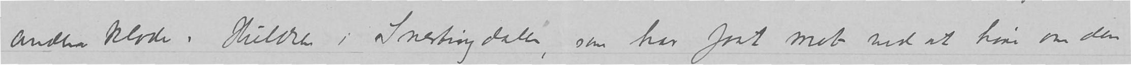anden klode: Huldren i Snertingdalen, som har faat mot ved at høre om den
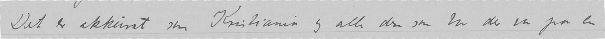Det er akkurat som Kristiania og alle dere som bor der var paa en
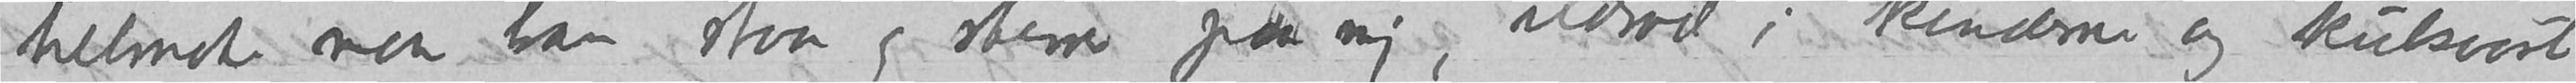til mote naar han staar og stirrer paa mig, ildrød i kinderne og kulsvart
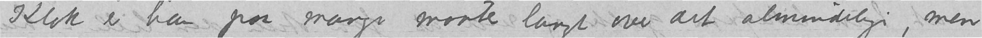Klok er han paa mange maater langt over det almindelige, men
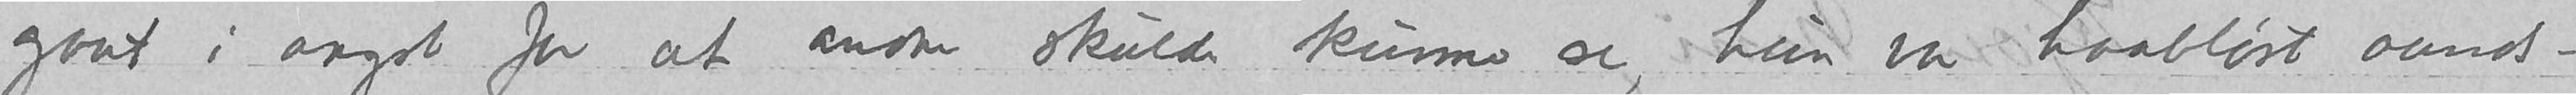gaat i angst for at andre skulde kunne se, hun var haabløst aands-
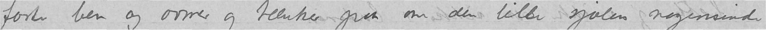forte ben og armer og tænker paa om den lille sjælen nogensinde
